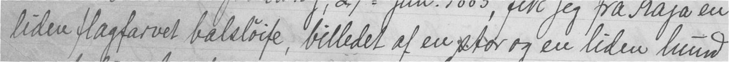liden flagfarvet balsløife, billedet af en stor og en liden hund
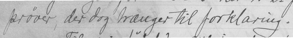prøver, der dog trænger til forklaring.
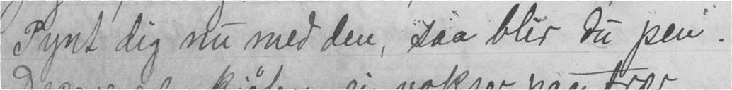Pynt dig nu med den, saa blir du pen.
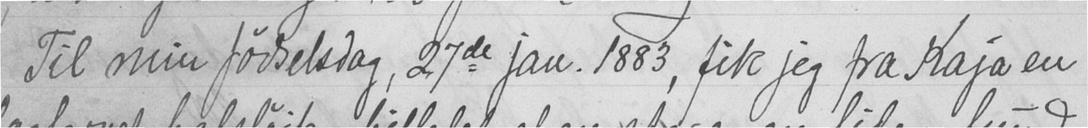Til min fødselsdag 27de jan. 1883, fik jeg fra Kaja en
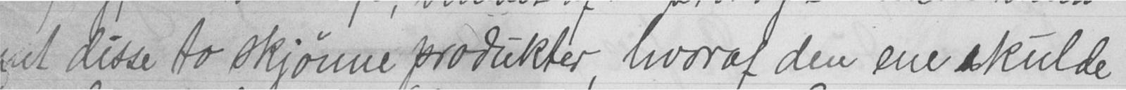mt disse to skjønne produkter, hvoraf den ene skulde
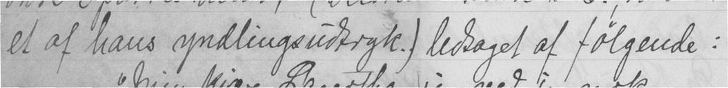et af hans yndlingsudtryk.) ledtaget af følgende:
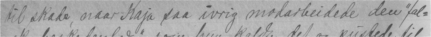til skade, naar Kaja saa ivrig modarbeidede den "fal-
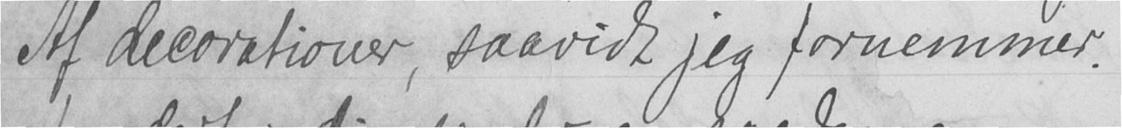Af decorationer, saavidt jeg fornemmer.
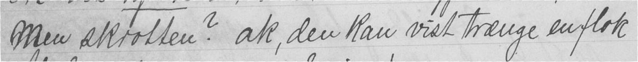men skrotten? ak, den kan vist trænge en flok
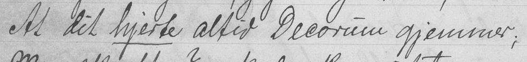At det hjerte altid Decorum gjemmer;
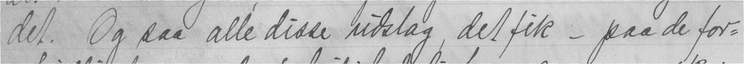det. Og saa alle disse udslag, det fik - paa de for-
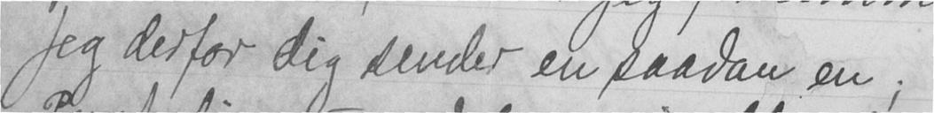Jeg derfor dig sender en saadan en;
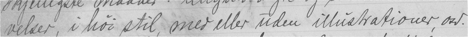velser, i høi stil, med eller uden illustrationer, osv.
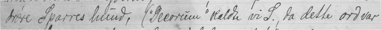være Sparres hund, ("Decorum" kaldte vi S., da dette ord var
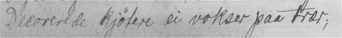Decorerede kjøtere ei vokser paa trær;
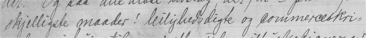skjelligste maader! leilighedsdigte og commerceskri-
